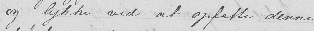og lykke ved at opfatte denne
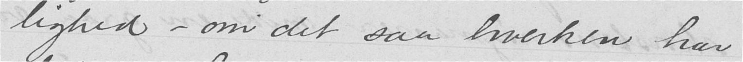lighed - om det saa hverken har
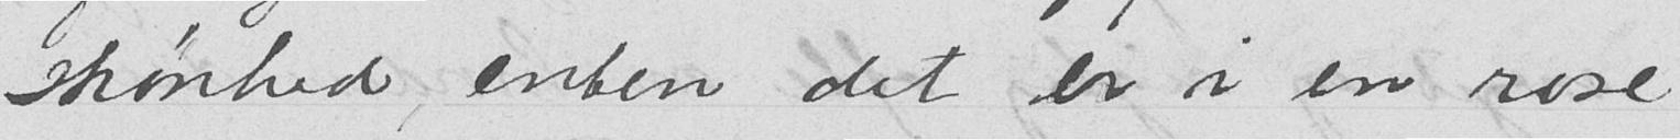skønhed, enten det er i en rose
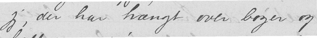jeg, der har hængt over bøger og
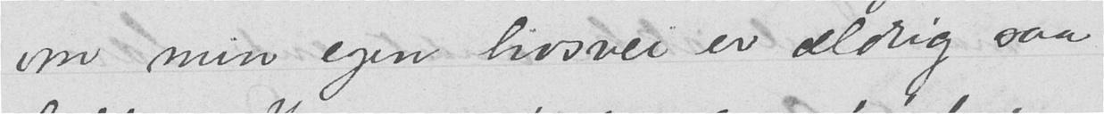om min egen livsvei er aldrig saa
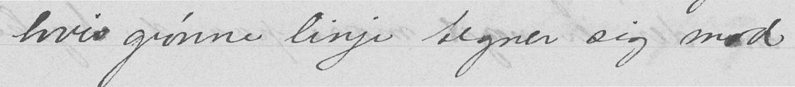hvis grønne linje tegner sig mod
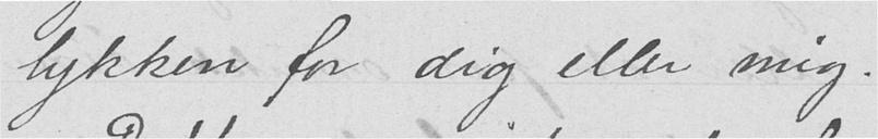lykken for dig eller mig.
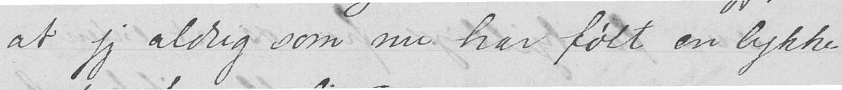at jeg aldrig som nu har følt en lykke
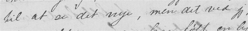til at se det nye, men det ved jeg,
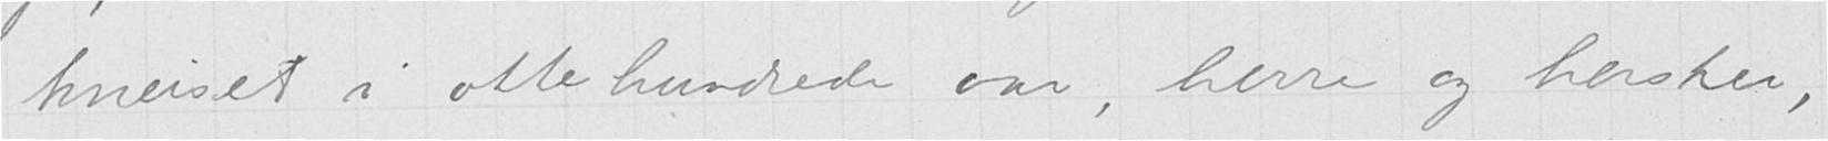kneiset i ottehundrede aar, herre og hersker,
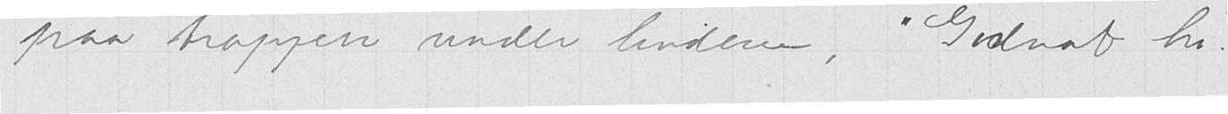paa trappen under linderne, "Godnat hr.
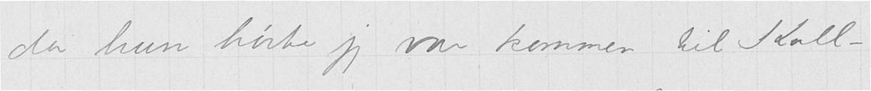da hun hørte jeg var kommen til Kall.
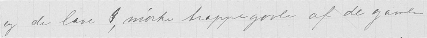og de lave , mørke trappegavler, af de gamle
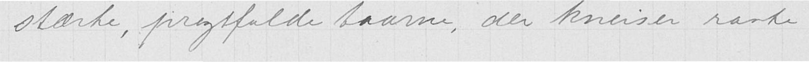stærke, pragtfulde taarne, der kneiser ranke
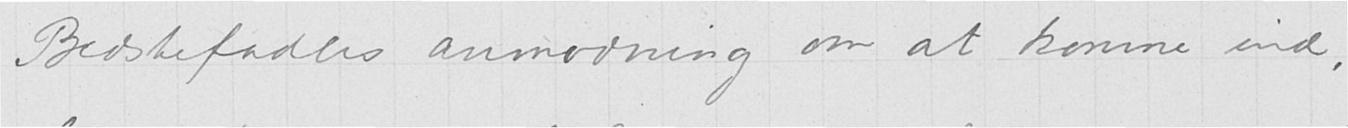Bedstefaders anmodning om at komme ind,
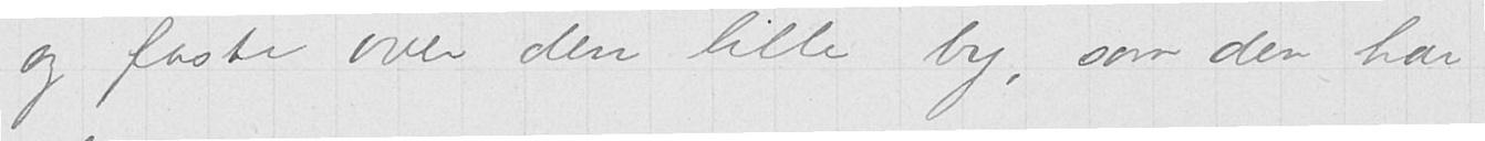og faste over den lille by, som den har
